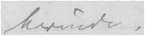herinde.
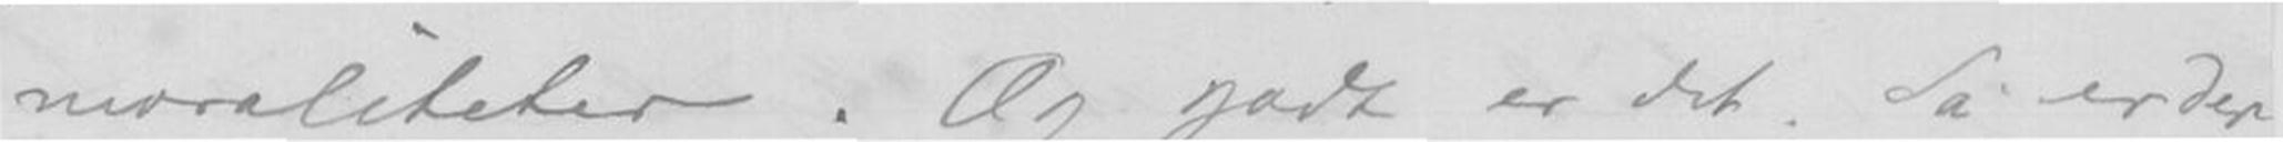moraliteter. Og godt er det. Så er der
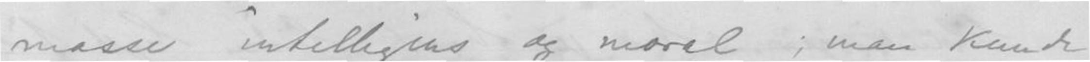masse intelligens og moral; man kunde
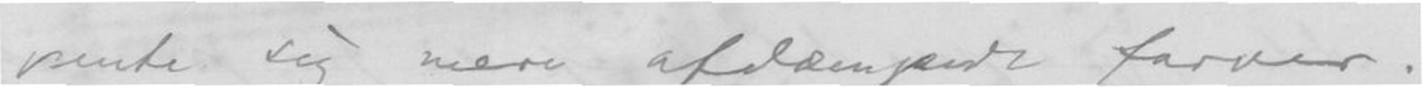vente sig mere afdæmpende farver.
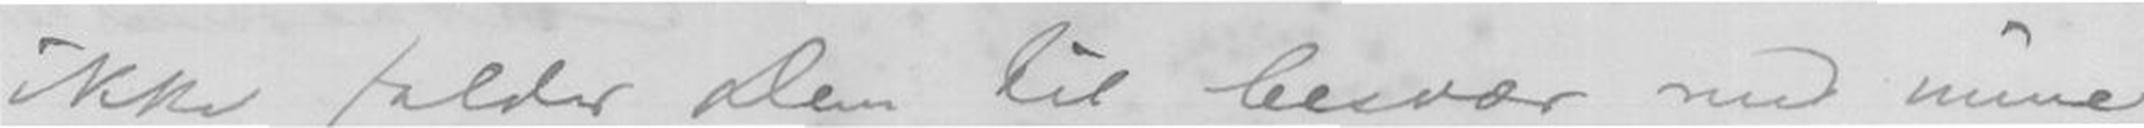ikke falder Dem til besvær med mine
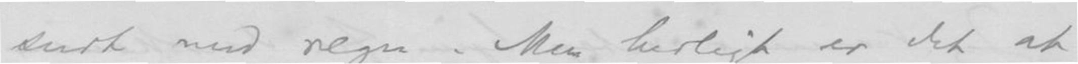surt med regn. Men herligt er det at
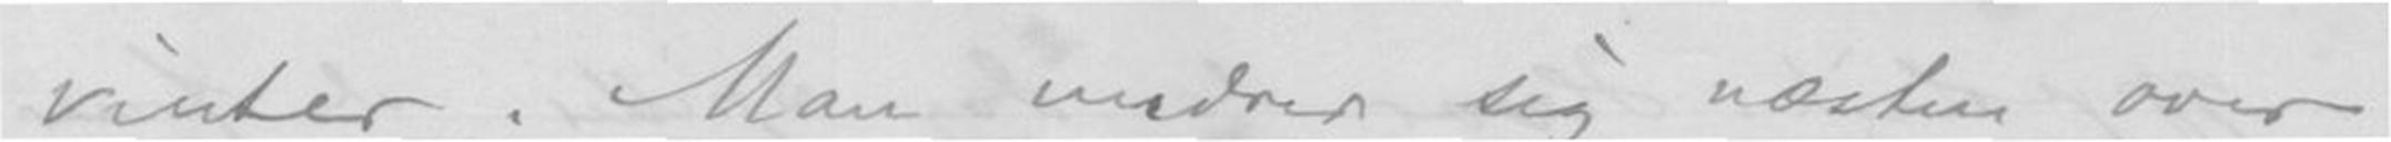vinter. Man undrer sig næsten over
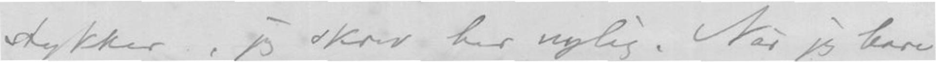jeg skrev her nylig. Når jeg bare
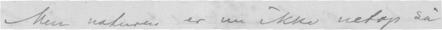Man naturen er nu ikke netop så
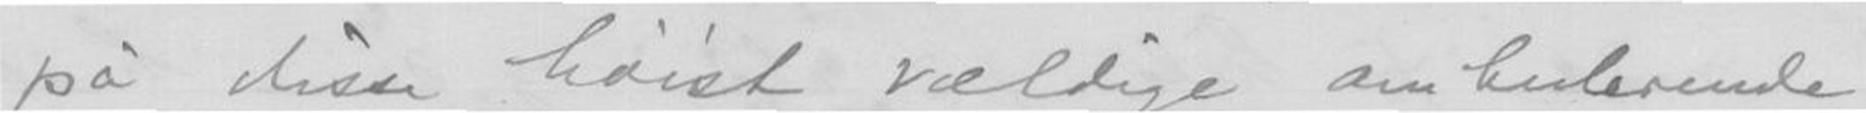på disse høist vældige ambulerende
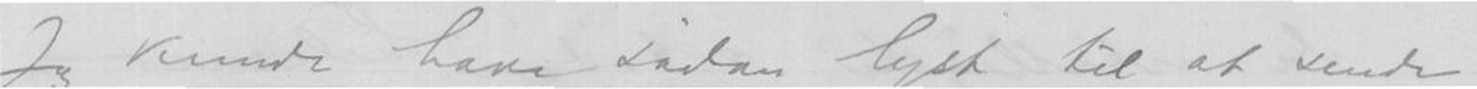Jeg kunde have sådan lyst til at sende
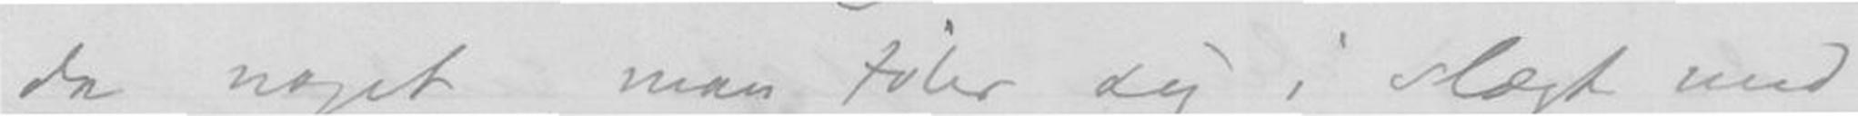da noget, man føler sig i slægt med
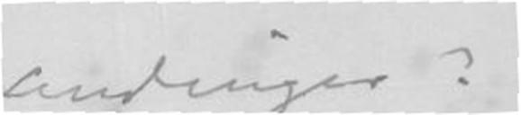sendinger?
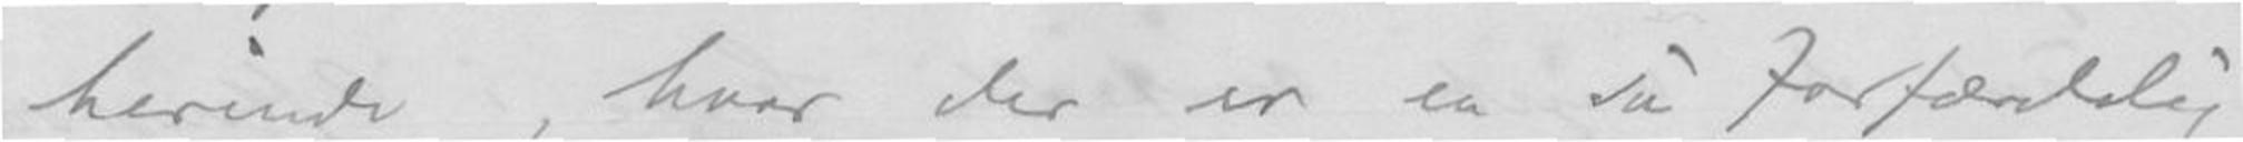herinde, hvor der er en så forfærdelig
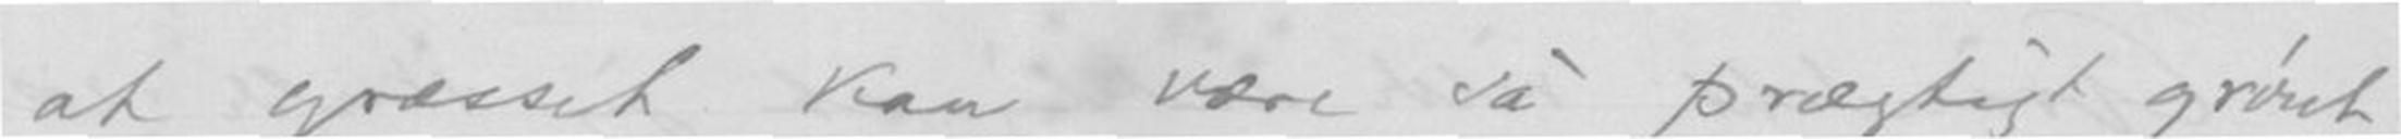at græssset kan være så prægtigt grønt
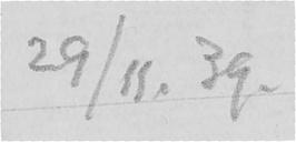29/11. 39.
